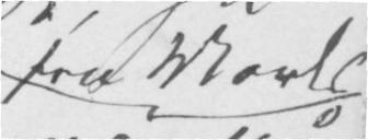fra Marts
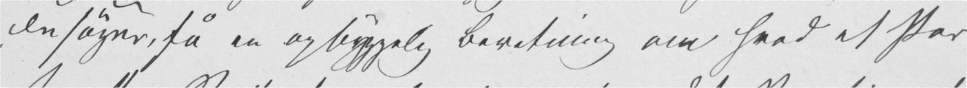Du søger, så en opbyggelig Beretning om hvad et Par
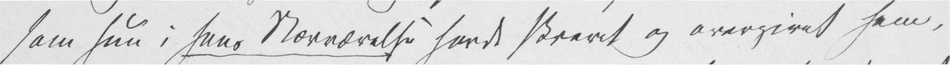som hun i hans Nærværelse havde skrevet og overgivet ham,
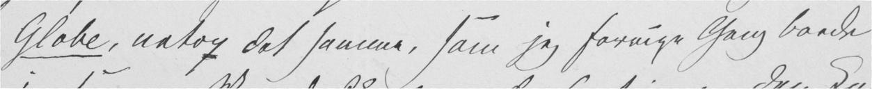Globe, netop det samme, som jeg forrige Gang boede
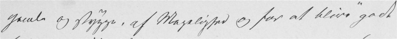gamle og stygge, af Magelighed og for at blive "godt
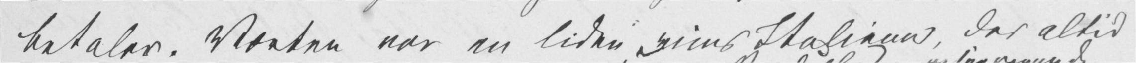betaler. Værten var en liden, vims Italiener, der altid
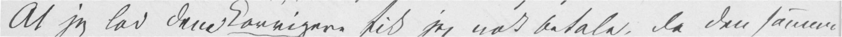At jeg lod den korrigere fik jeg nok betale, da den samme
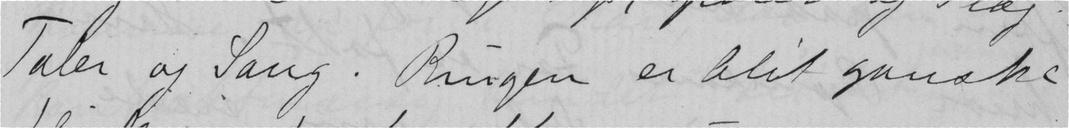Taler og Sang. Ringen er blit ganske
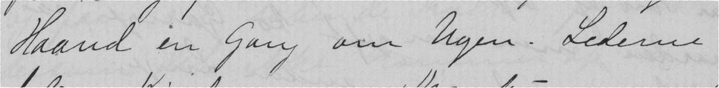Haand en Gang om Ugen. Lederne
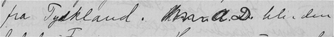fra Tyskland.  A. D. blir den
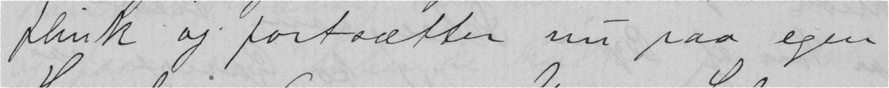flink og fortsætter nu paa egen
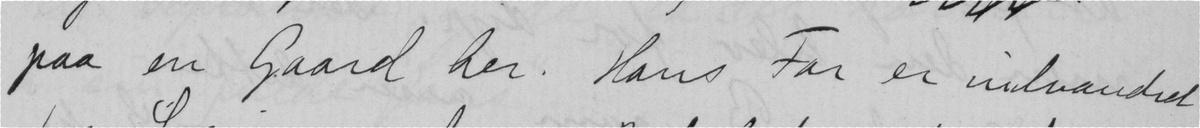paa en Gaard her. Hans Far er indvandret
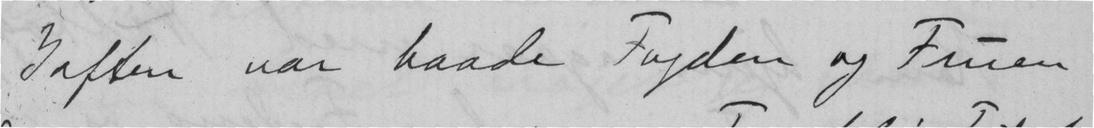Iaften var baade Fogden og Fruen
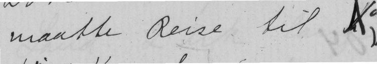maatte Reise til
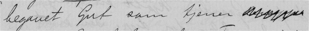begavet Gut som tjener
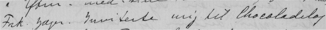Frk. Jæger. Inviterte mig til Chocoladelag
